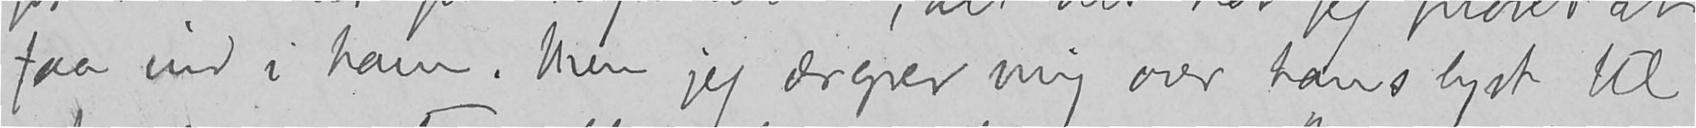faa ind i ham. Men jeg ærgrer mig over hans lyst til
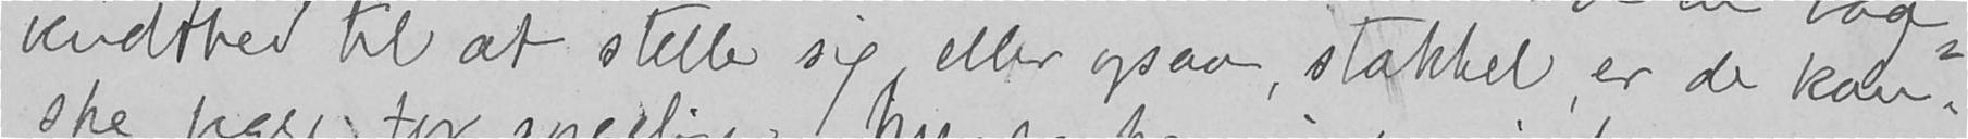vendthed til at stille sig, eller ogsaa, stakkel, er de kan-
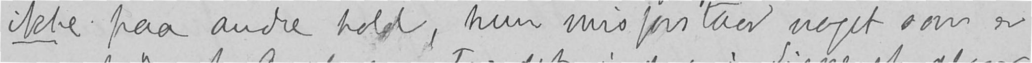ikke paa andre hold, hun misforstaar noget som er
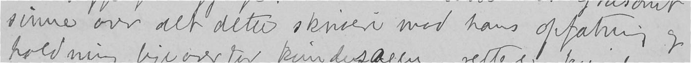sinne over alt dette skriveri mod hans opfatning og
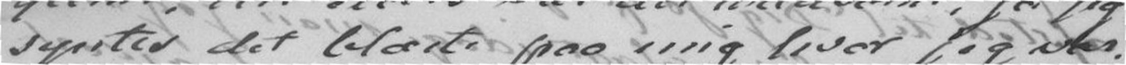syntes det blæste paa mig hvor jeg var,
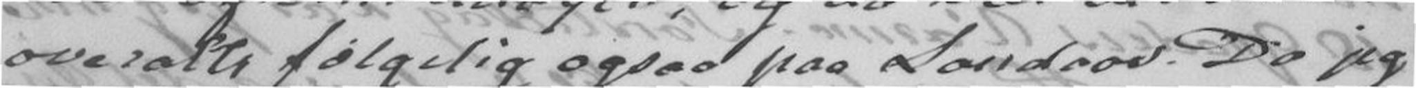overalt, følgelig ogsaa paa Landaas. Da jeg
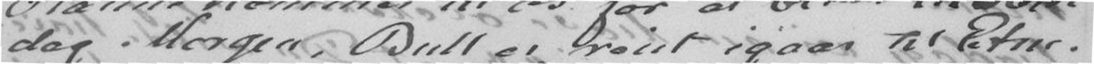dag Morgen. Bull er reist igaar til Etne.
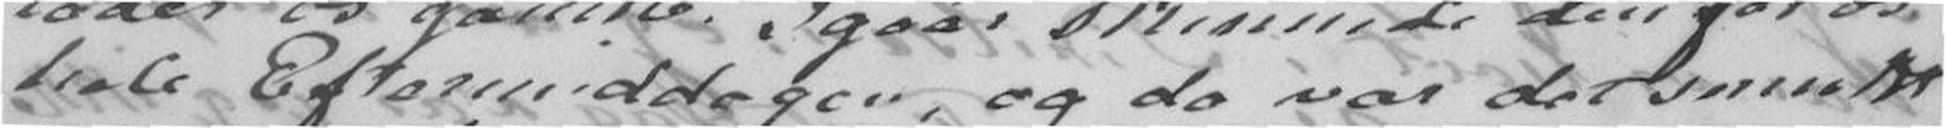hele Eftermiddagen, og da var det smukt
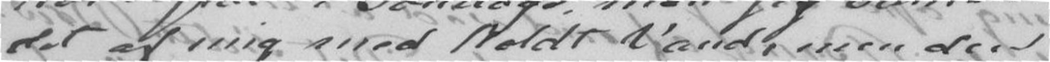det af mig med koldt Vand, men den
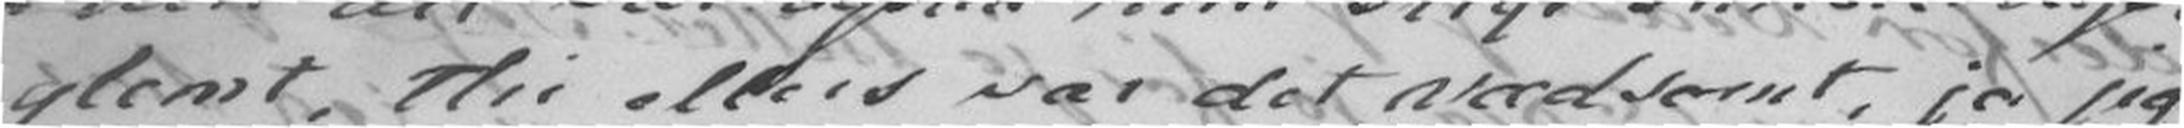glimt, thi ellers var det rædsomt, ja jeg
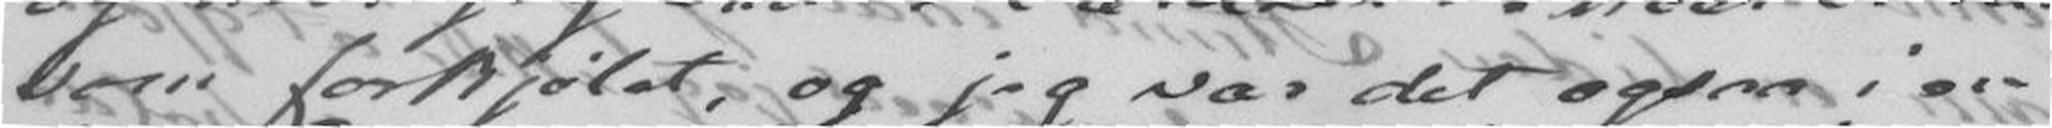som forkjølet, og jeg var det ogsaa i en
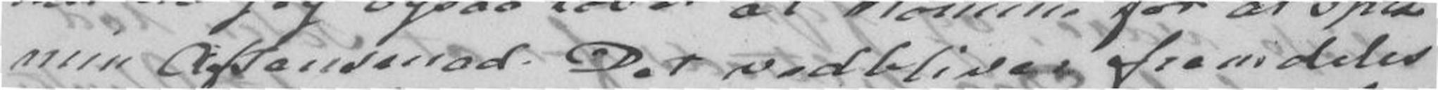min Aftensmad. Det vedbliver fremdeles
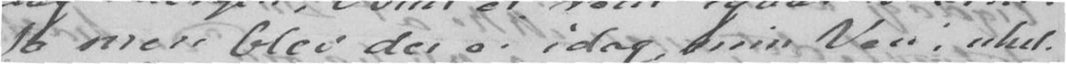Ja mere blev det ei idag, min Ven! uhel-
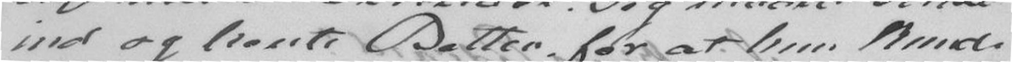ind og hente Betten, for at hun kunde
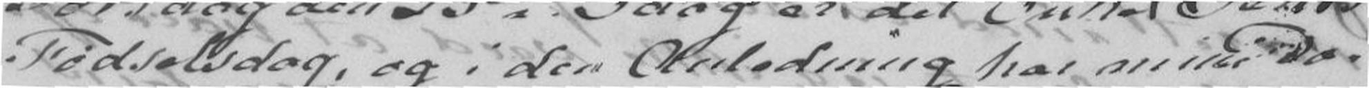Fødselsdag, og i den Anledning har mine Da-
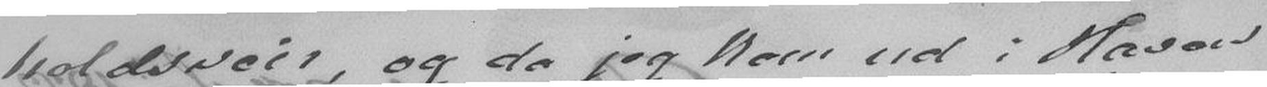holdsveir, og da jeg kom ud i Haven
